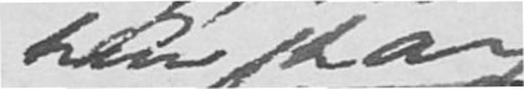hun har
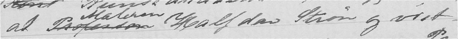at Professor Halfdan Strøm og vist-
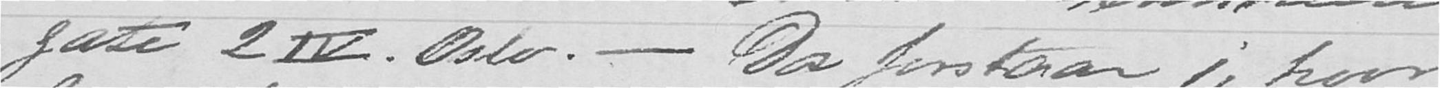gate 2 IV. Oslo. - Da forstaar jeg hvor
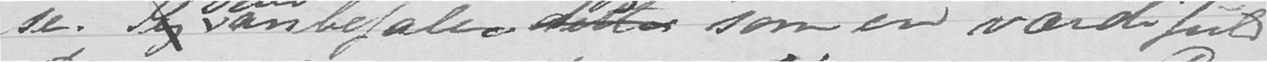se. Jeg anbefaler dette som en værdifuld
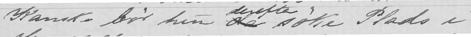Kanske bør hun da søke Plads i
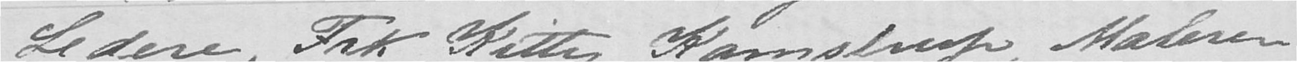Ledere, Frk Kitty Kamstrup Maleren
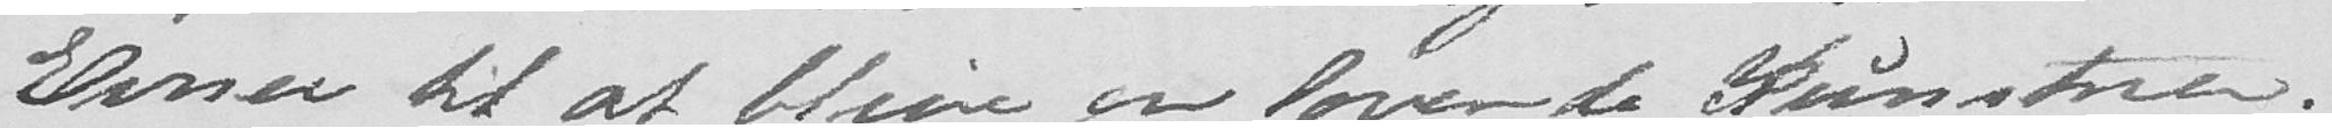Evner til at blive en lovende Kunstner.
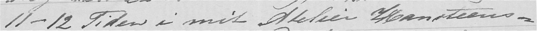11-12 Tiden i mit Atelier Hansteens-
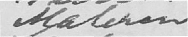Maleren
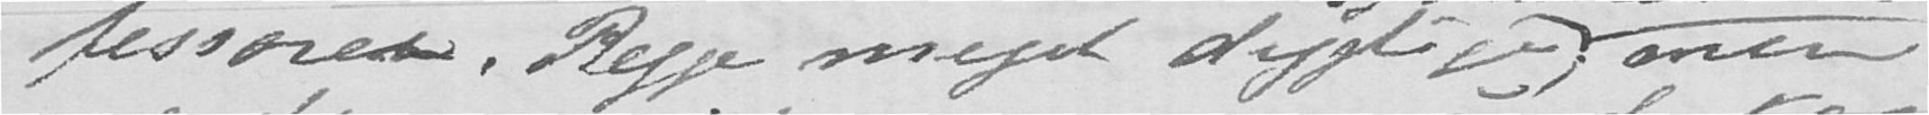fessoren. Begge meget dygtige; men
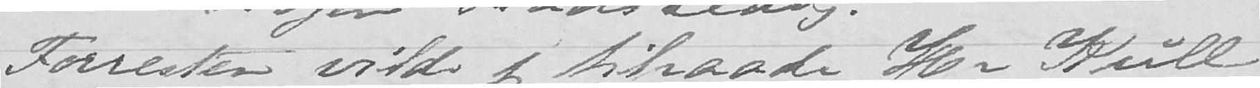Forresten vilde jeg tilraade Hr Kull
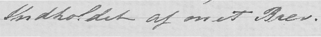Indholdet af mit Brev.
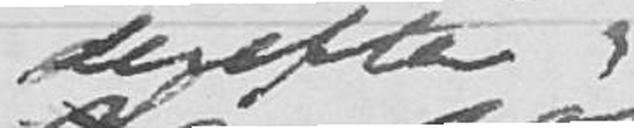derefter
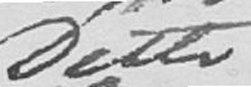Dette
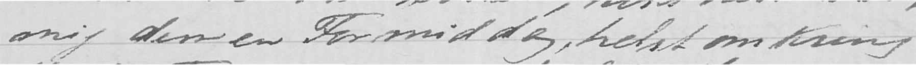mig dem en Formiddag, helst omkring
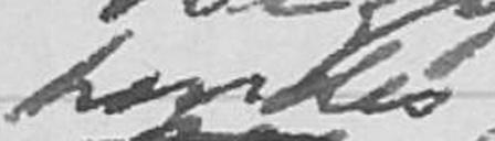hendes
